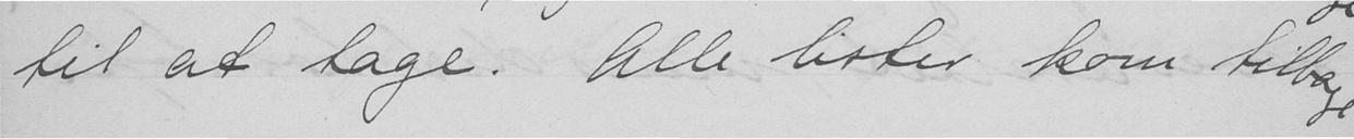til at tage. Alle lister kom tilbage
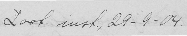Zoot. inst., 29-9-04.
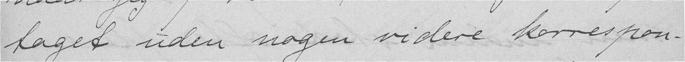taget uden nogen videre korrespon-
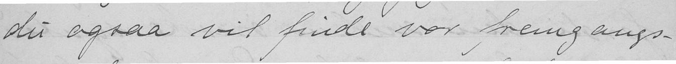du ogsaa vil finde vor fremgangs-
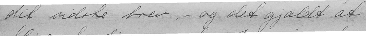det sidste brev, - og det gjaldt at
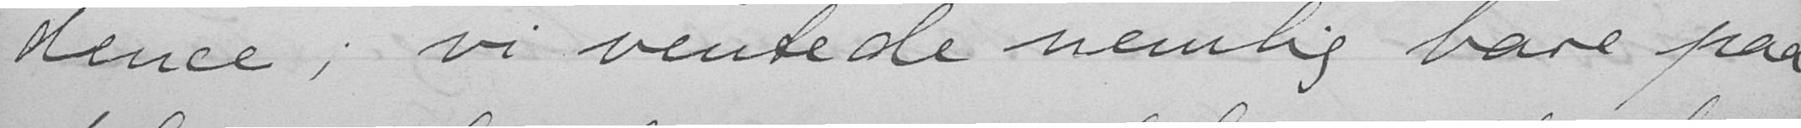dence; vi ventede nemlig bare paa
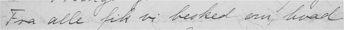Fra alle fik vi besked om, hvad
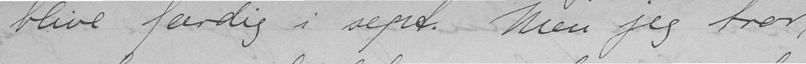blive færdig i sept. Men jeg tror,
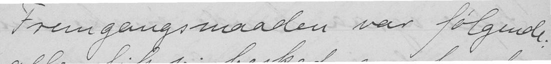Fremgangsmaaden var følgende:
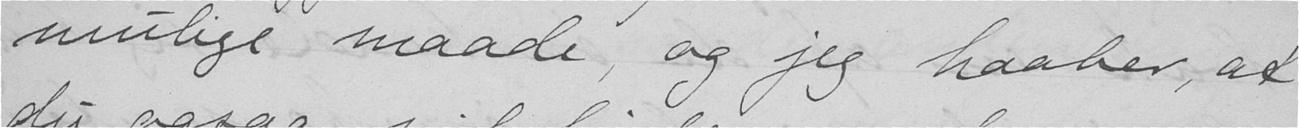mulige maade, og jeg haaber at
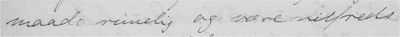maade rimelig og være tilfreds
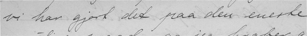vi har gjort det paa den eneste
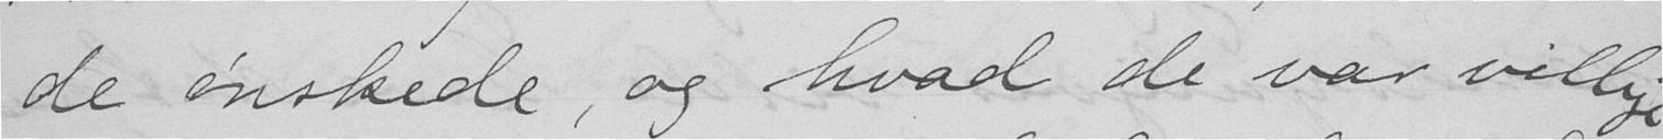de ønskede, og hvad de var villige
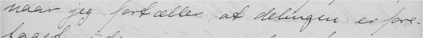naar jeg fortæller, at delingen er fore-
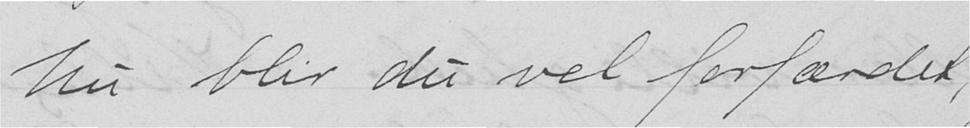Nu blir du vel forfærdet,
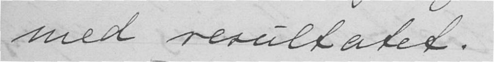med resultatet.
